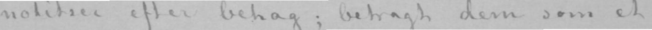notitser efter behag; betragt dem som et
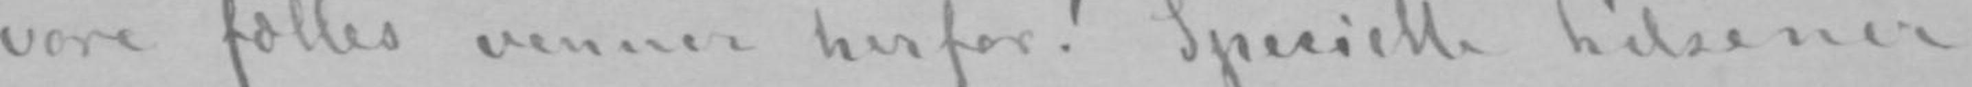vore fælles venner herfor! Specielle hilsener
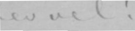Lev vel!
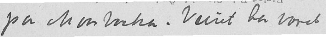paa Maarbacka. Veiret har været
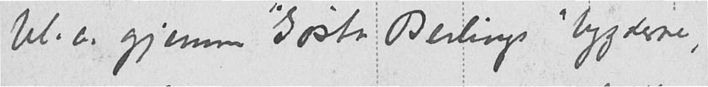bl.a. gjennem "Gøsta Berlings" bygderne,
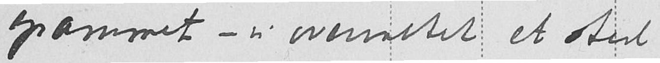grammet – vi overnattet et sted
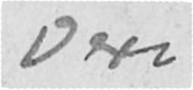Deres
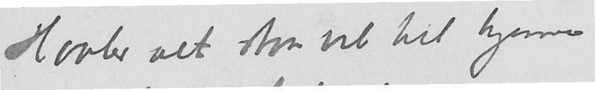Haaber alt staar vel til hjemme
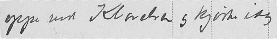oppe ved Klarelven og kjørte idag
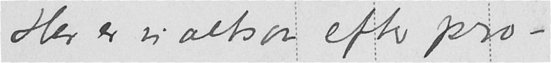Her er vi altsaa efter pro-
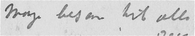Mange hilsener til alle
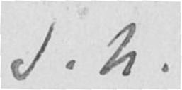S.U.
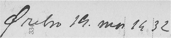Ørebro 19. mai 1932
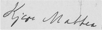Kjære Mathea
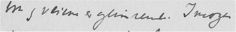bra og veiene er glimrende. I morgen
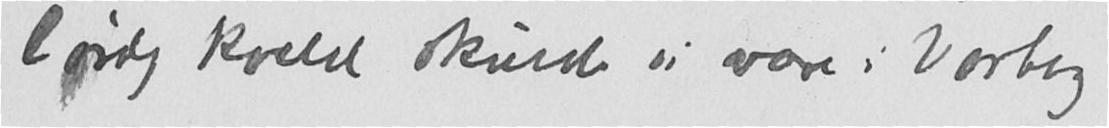lørdag kveld skulde vi være i Varberg
